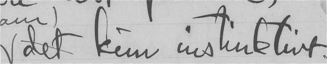det kun instinktivt,
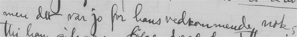men det var jo for hans vedkommende nok; -
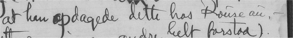at han opdagede dette hos Rouseau, -
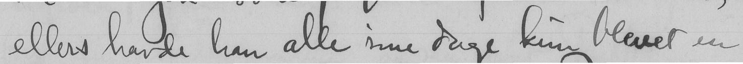ellers havde han alle sine dage kun blevet en
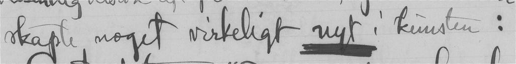skapte noget virkeligt nyt i kunsten:
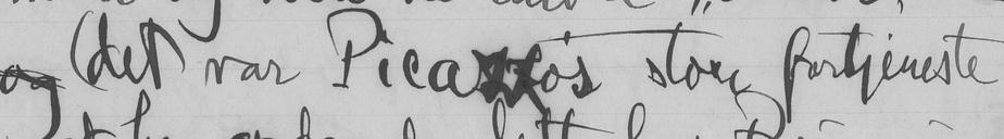og (det var Picassos store fortjeneste
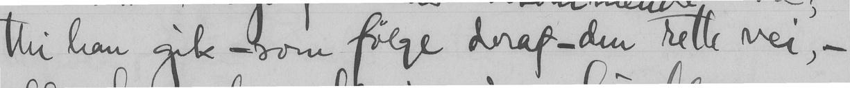thi han gik -som følge deraf- den rette vei, -
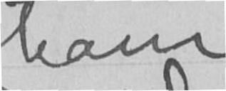ham
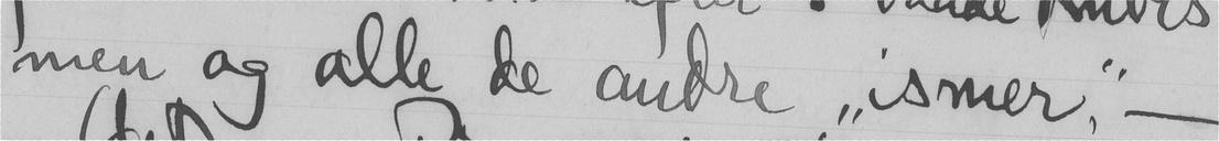men og alle de andre "ismer", -
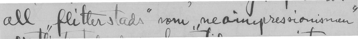all "flitterstads" som "neoimpressionismen"
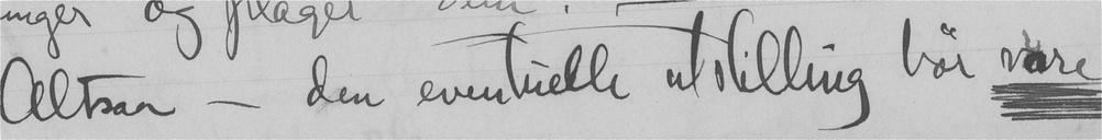Altsaa - den eventuelle utstilling bør vøre
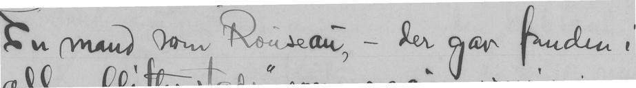En mand som Rouseau - der gav fanden i
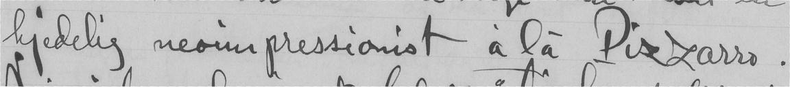kjedelig neoimpressionist àla Picasso.
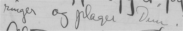ringer og plager Dem.
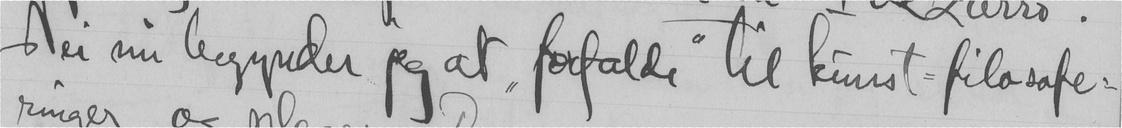Nei nu begynder jeg at "forfalde" til kunst-filosofe-
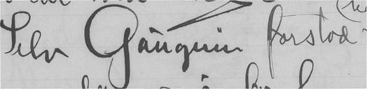Selv Gauguin forstod
